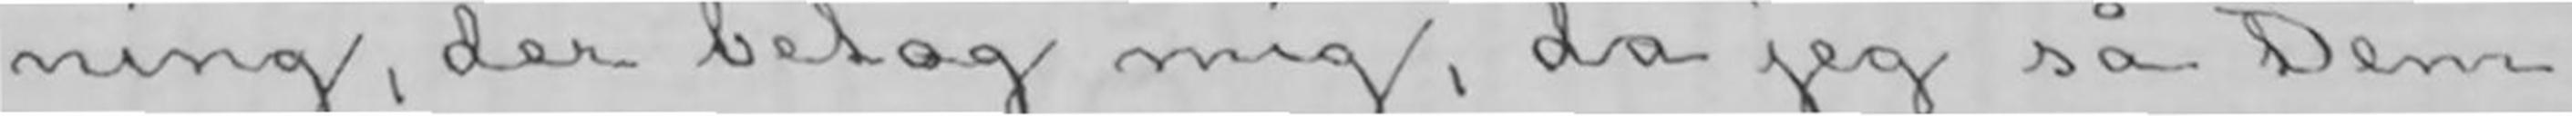ning, der betog mig, da jeg så Dem
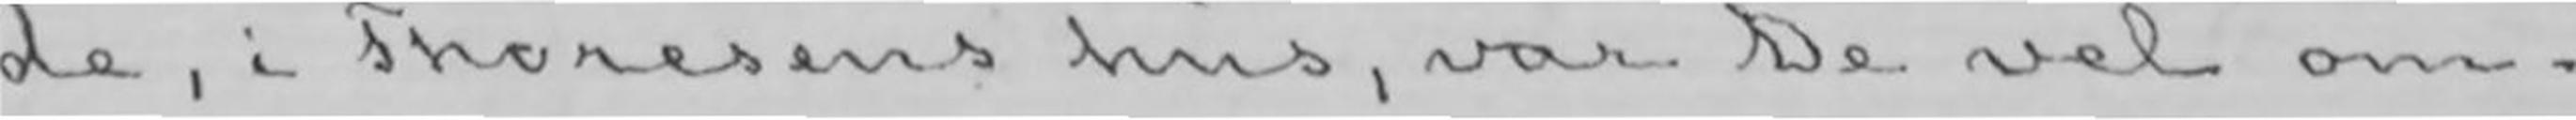de, i Thoresens hus, var De vel om-
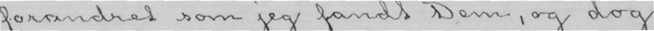forandret som jeg fandt Dem, og dog
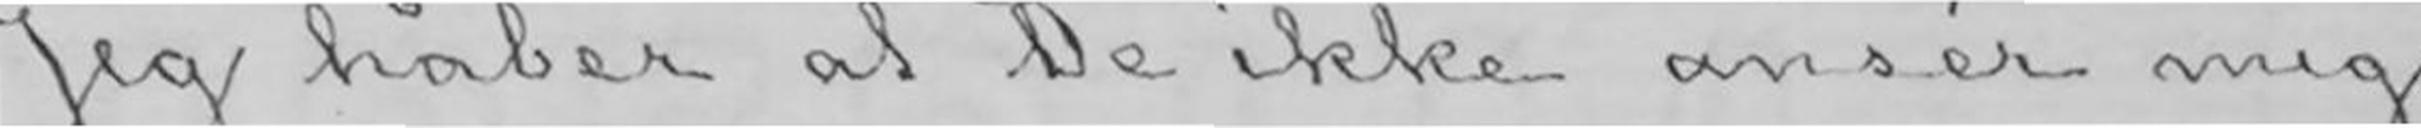Jeg håber at De ikke ansér mig
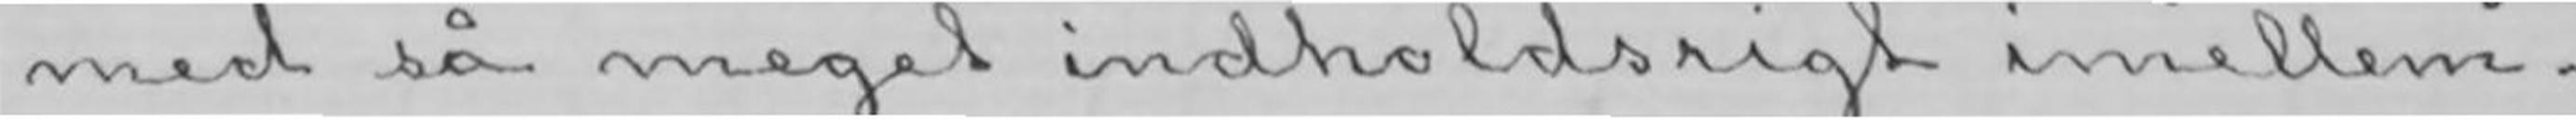med så meget indholdsrigt imellem.
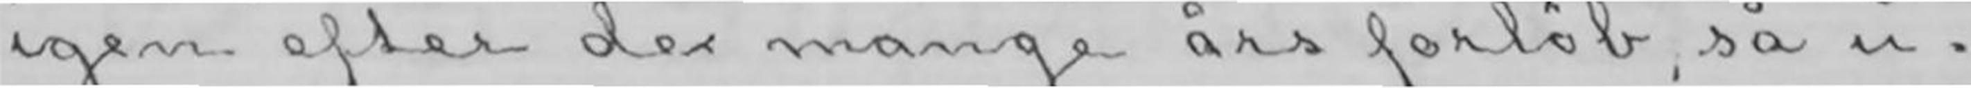igen efter de mange års forløb, så u-
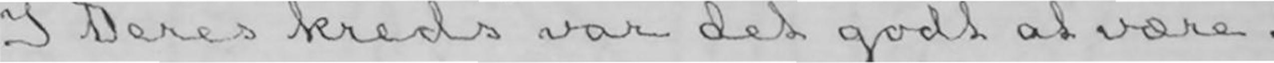I Deres kreds var det godt at være.
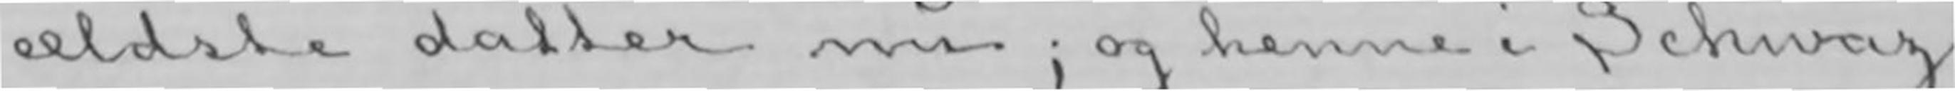ældste datter nu; og henne i Schwaz
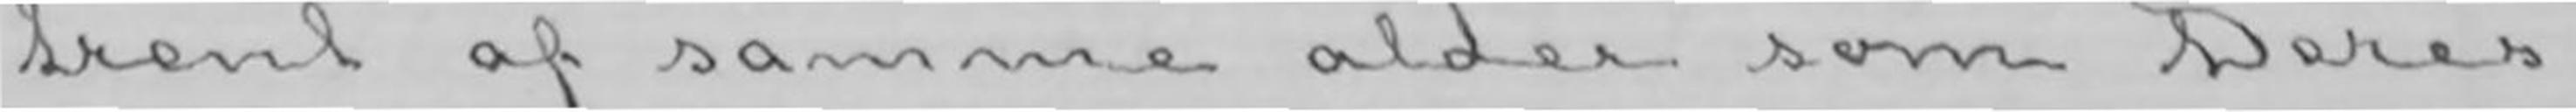trent af samme alder som Deres
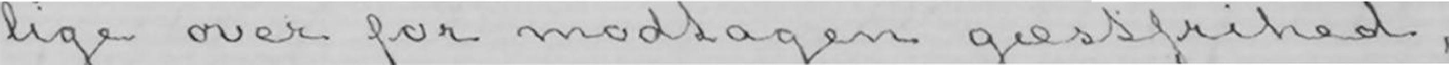lige over for modtagen gæstfrihed,
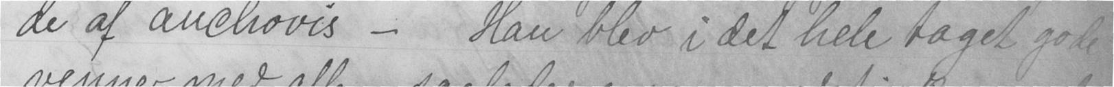de af anchovis - Han blev i det hele taget gode
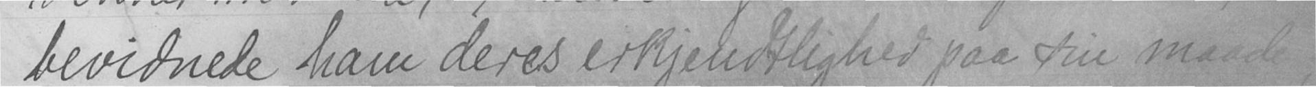bevidnede ham deres erkjendtlighed paa sin maade;
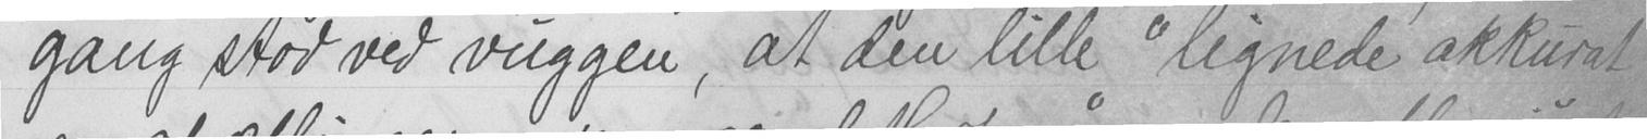gang stod ved vuggen, at den lille "lignede akkurat,"
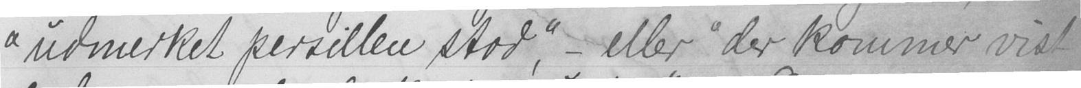"udmerket persillen stod", - eller "der kommer vist
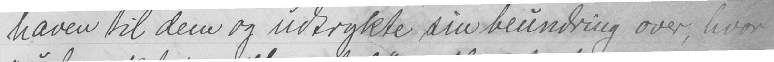haven til dem og udtrykte sin beundring over, hvor
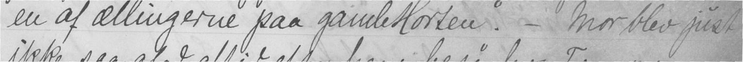en af ællingerne paa gamleHorten." - Mor blev just
